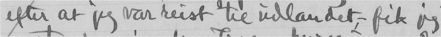efter at jeg var reist til udlandet, fik jeg
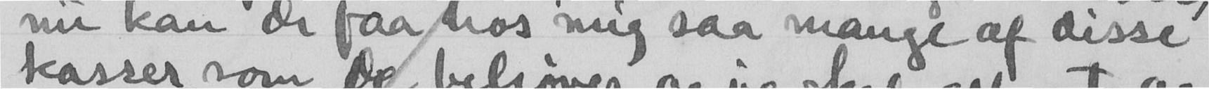nu kan de faa hos mig saa mange af disse
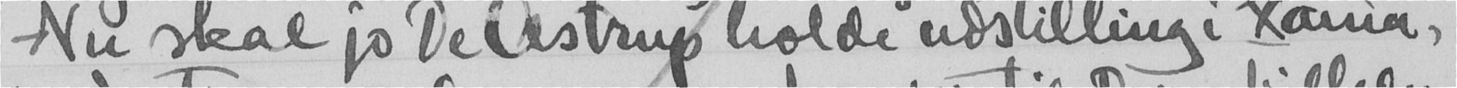Nu skal jo De Astrup holde udstilling i Xania,
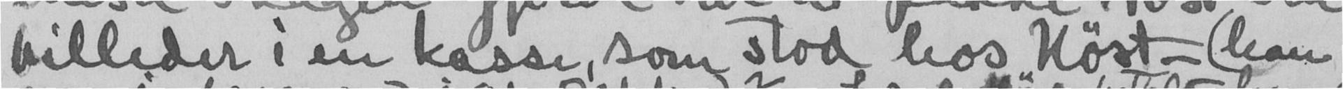billeder i en kasse, som stod hos Høst,- (han
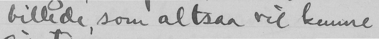billede, som altsaa vil kunne
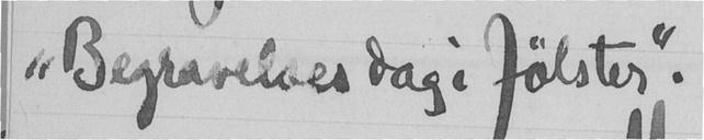"Begravelses dag i Jølster".
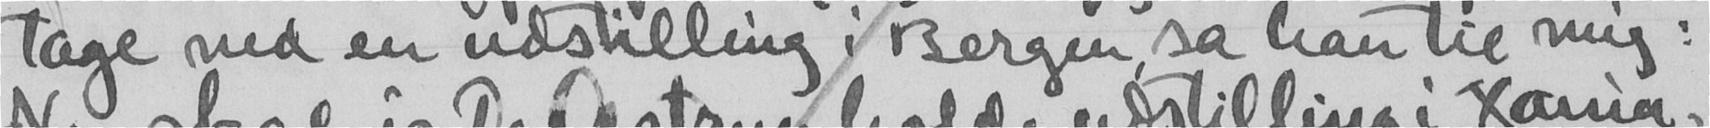tage ned en udstilling i Bergen sa han til mig:
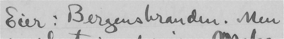Eier: Bergensbranden. Men
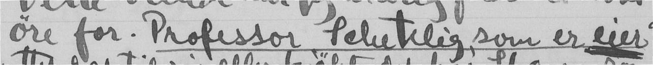øre for. Professor Schetelig, som er eier
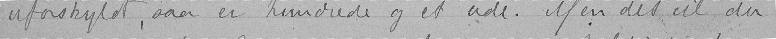uforskyldt, saa er hundrede og et ude. Men det vil du
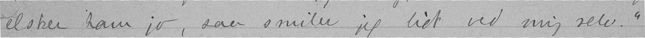elsker ham jo, saa smiler jeg lidt ved mig selv."
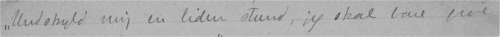"Undskyld mig en liden stund, jeg skal bare give
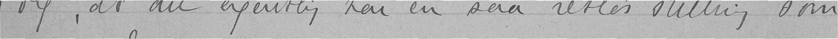dig, at du egentlig har en saa retløs stilling som
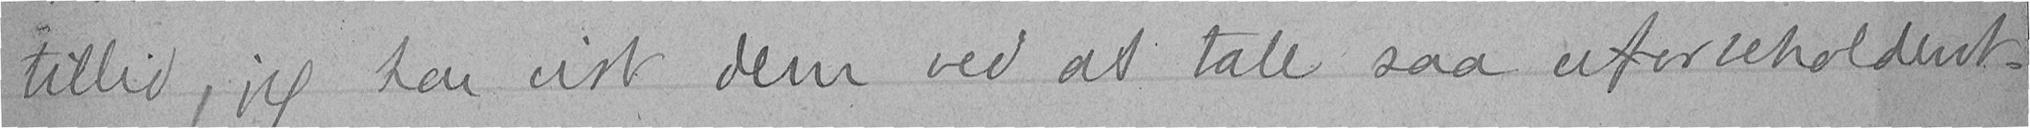tillid, jeg har vist dem ved at tale saa uforbeholdent.
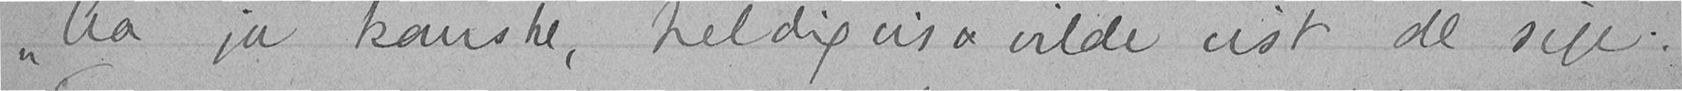"Aa ja kanske, heldigvis vilde vist de sige.
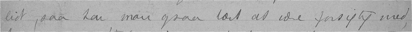lidt, saa har man ogsaa lært at være forsigtig med,
